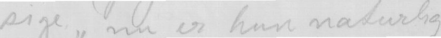sige nu er hun naturlig-
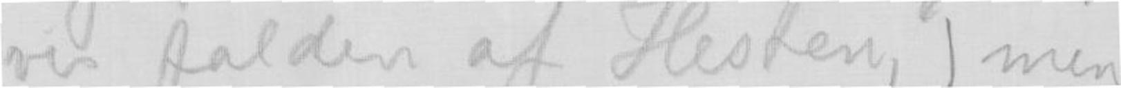vis falden af Hesten,) men
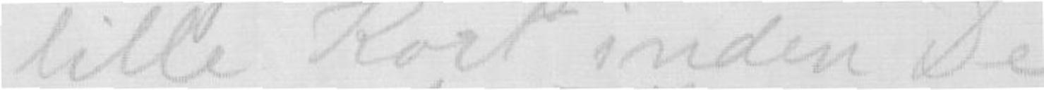lille Kort inden De
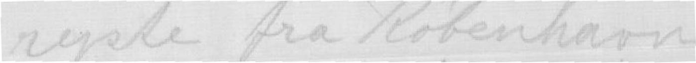rejste fra København,
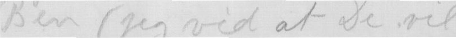ben (jeg ved at De vil
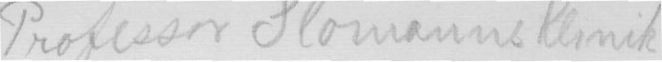Professor Slomannsklinik
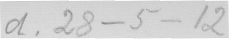d.28-5-12
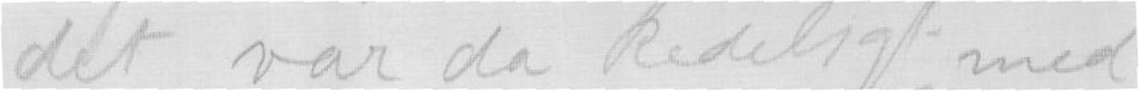det var da kedeligt med
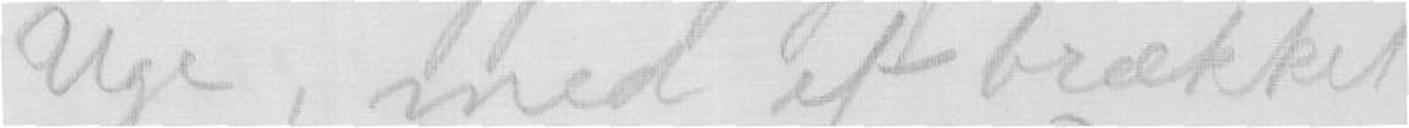Uge, med et brækket
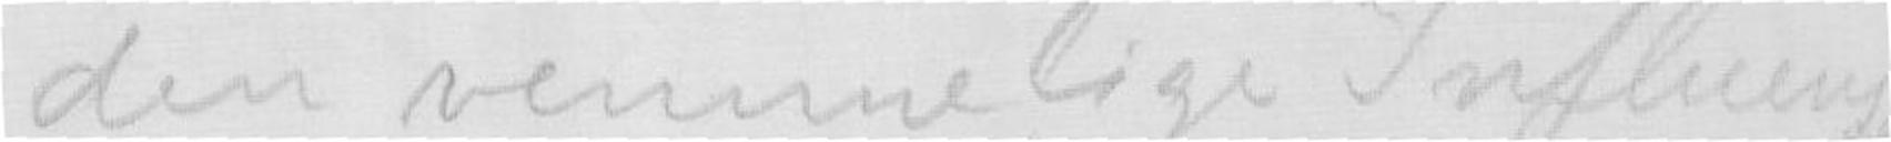den vemmelige Influenza
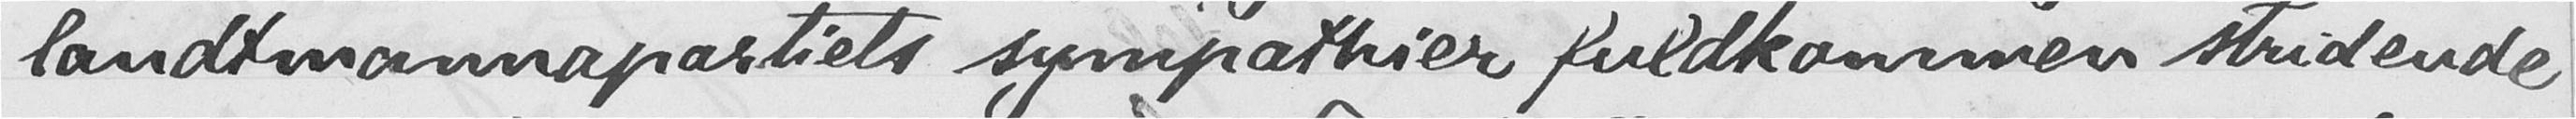landtmannapartiets sympathier fuldkommen stridende
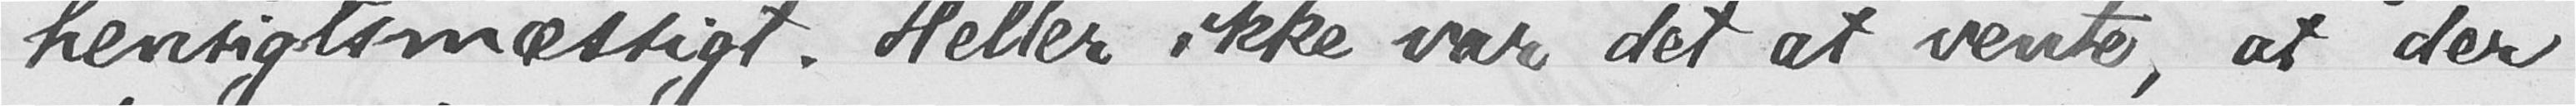hensigtsmæssigt. Heller ikke var det at vente, at der
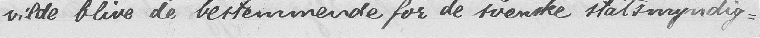vilde blive de bestemmende for de svenske statsmyndig-
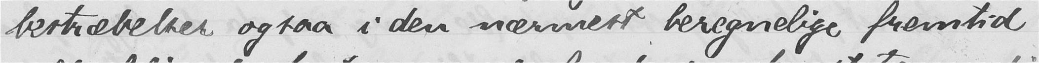bestræbelser ogsaa i den nærmest beregnelige fremtid
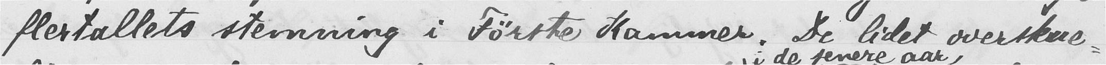flertallets stemning i Første Kammer. De lidet overskue-
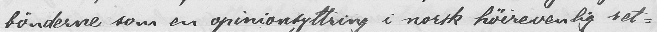bønderne som en opinionsyttring i norsk høirevenlig ret-
